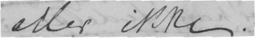eller ikke.
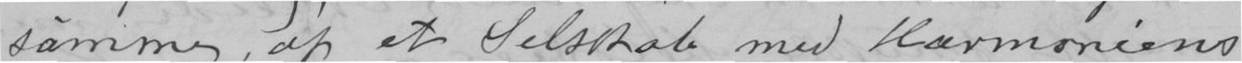samme, af et Selskab med Harmoniens
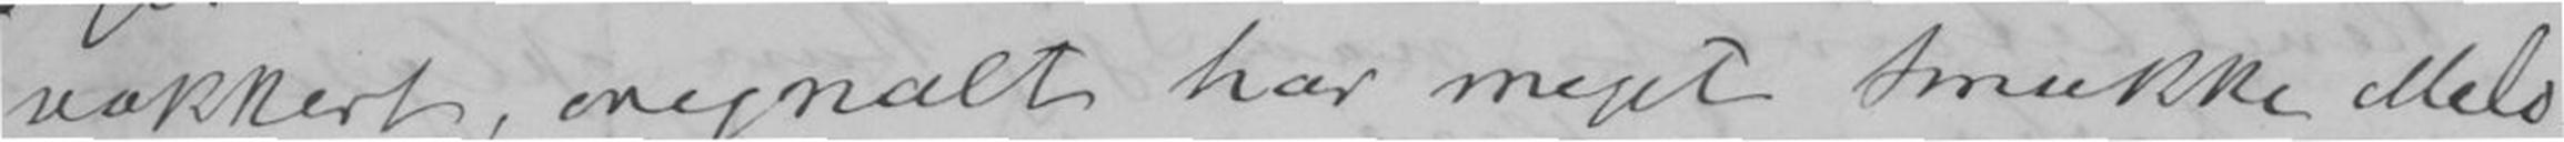vakkert, originalt har meget smukke Melo-
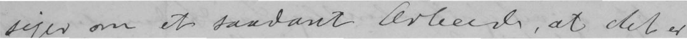siger om et saadant Arbeide, at det er
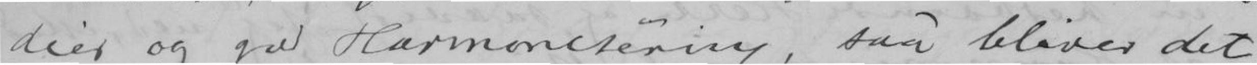dier og god Harmonisering, saa bliver det
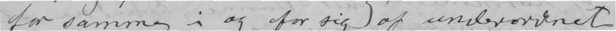for samme i og for sig af underordnet
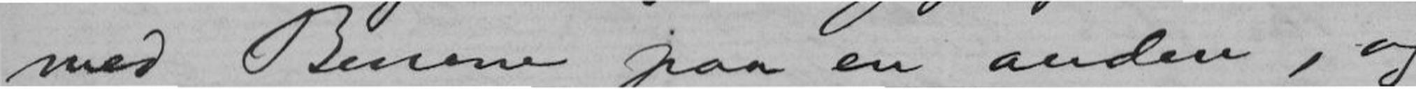med Benene paa en anden, og
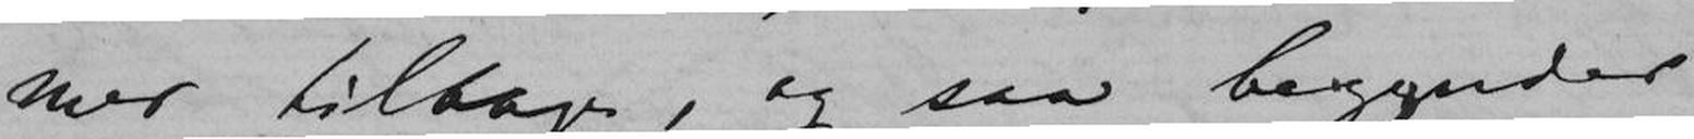mer tilbage, og saa begynder
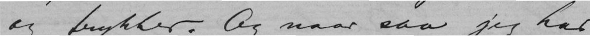og trykker. Og naar saa jeg har
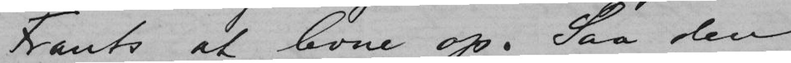Frants at levne op. Saa den
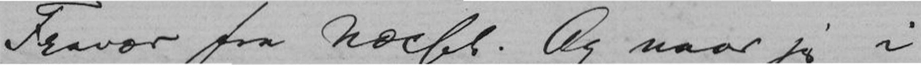Fravær fra Næsset. Og naar jeg i
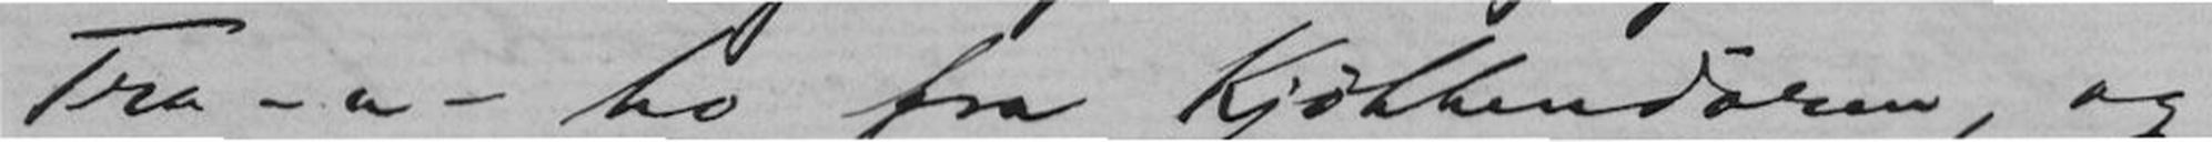Tra-a-ho fra Kjøkkendøren, og
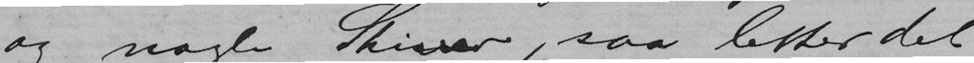og nogle Skiver, saa letter det
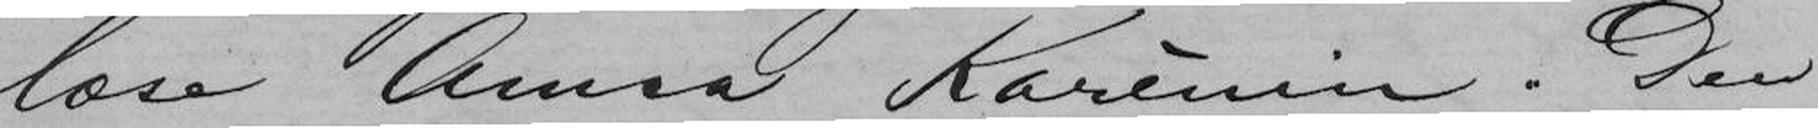læse Anna Karènin. Den
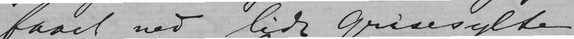faaet ned lidt Grisesylte
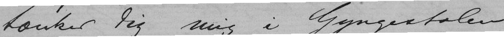tænker Dig mig i Gyngestolen
